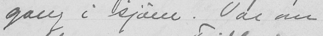gang i sjøen. Var om
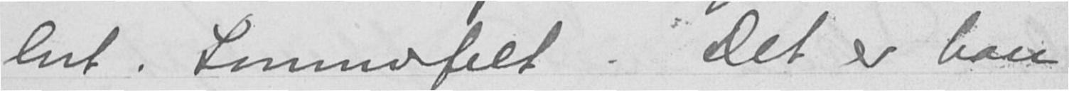lnt. Sommerfelt. Det er han
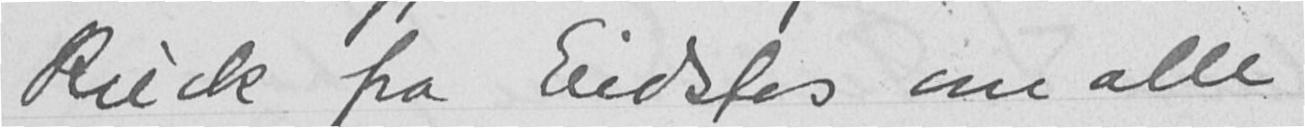Rieck fra Eidsfos om alle
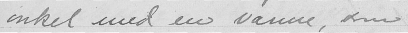onkel med en varme, som
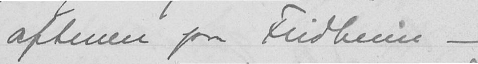aftenen paa Fridheim -
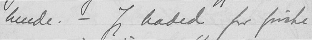hende. - Jeg haded for første
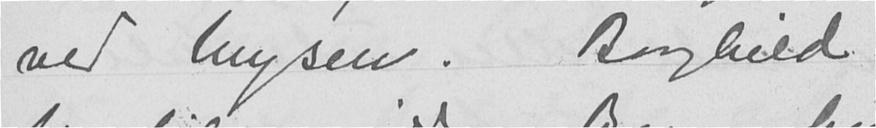ved Mysen. Borghild
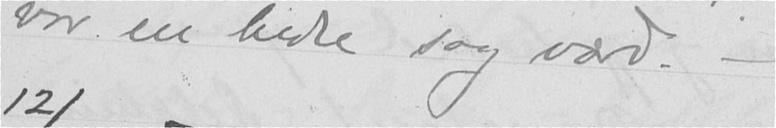var en bedre sag værd. -
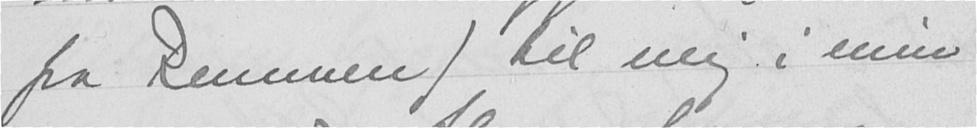fra Remmen) til mig i min
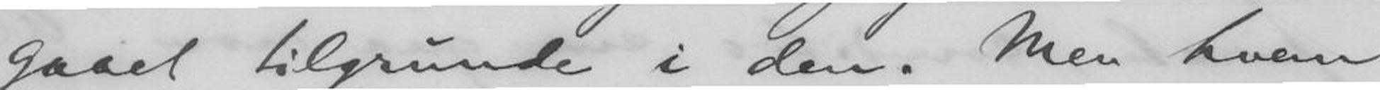gaaet tilgrunde i den. Men hvem
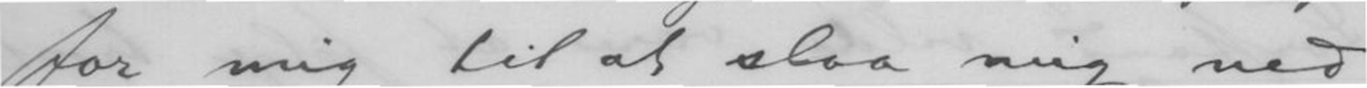for mig til at slaa mig ned
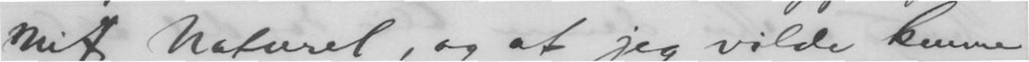mit Naturel, og at jeg vilde kunne
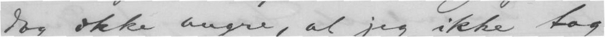dog ikke angre, at jeg ikke tog
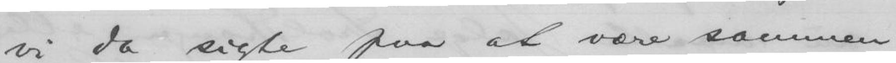vi da sigte paa at være sammen
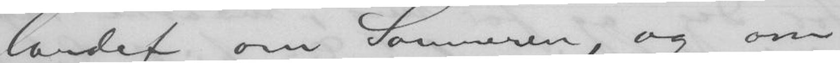landet om Sommeren, og om
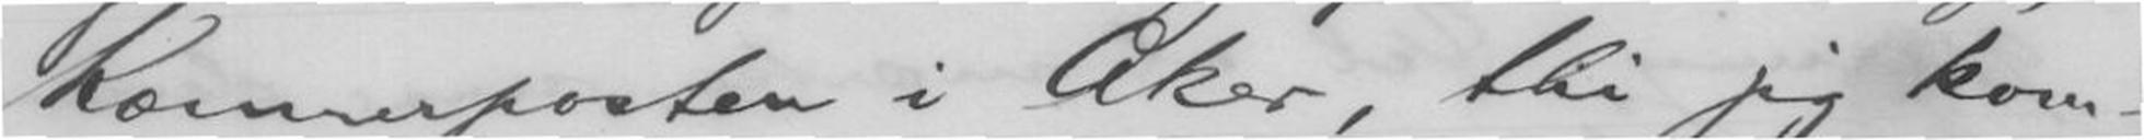Kæmnerposten i Aker, thi jeg kom-
